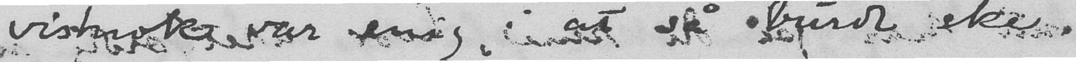vistnok var enig i at så burde ske.
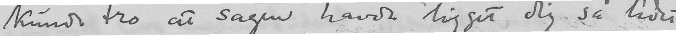kunde tro at sagen havde ligget dig så lidet
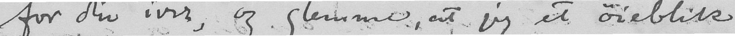for din iver, og glemme, at jeg et øieblik
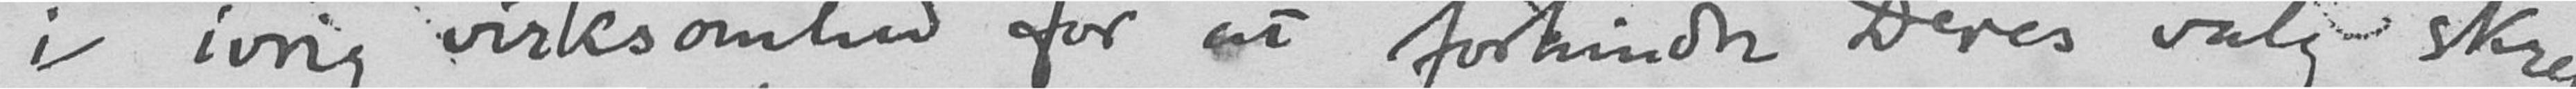i ivrig virksomhed for at forhindre Deres valg" skrev
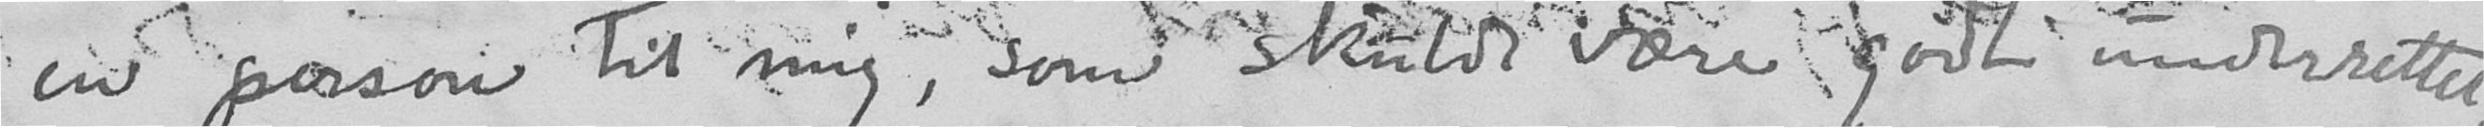en person til mig, som skulde være godt underrettet;
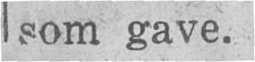som gave.
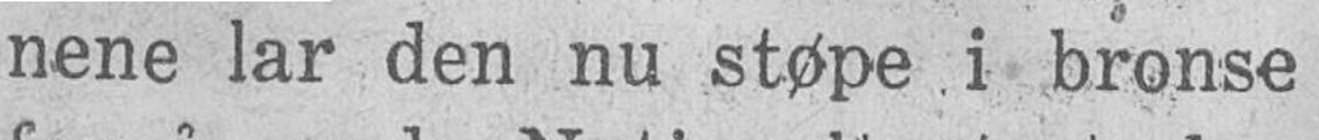nene lar den nu støpe i bronse
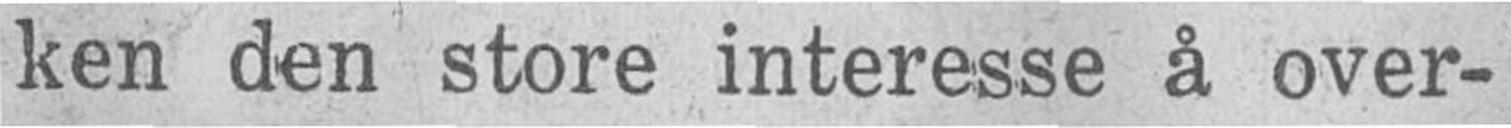ken den store interesse å over-
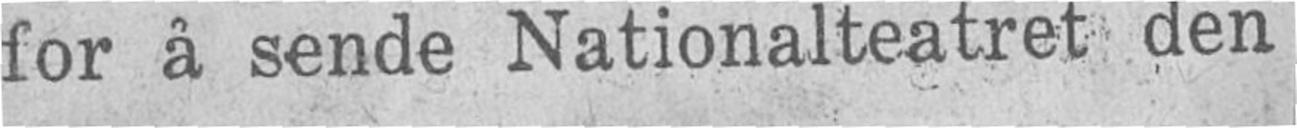for å sende Nationalteatret den
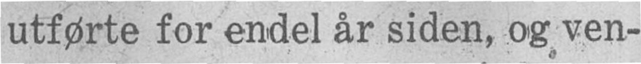utførte for endel år siden, og ven-
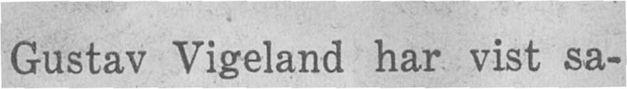Gustav Vigeland har vist sa-
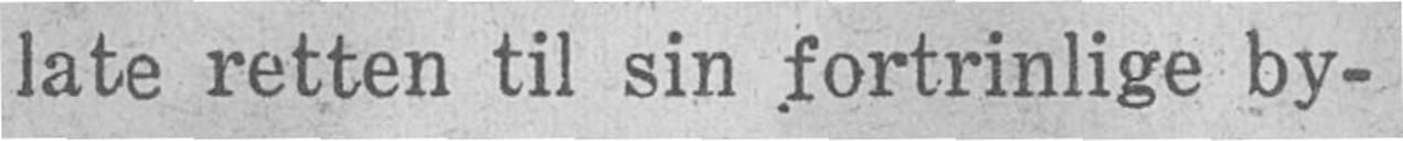late retten til sin fortrinlige by-
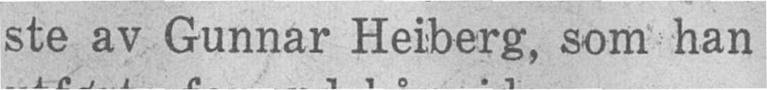ste av Gunnar Heiberg, som han
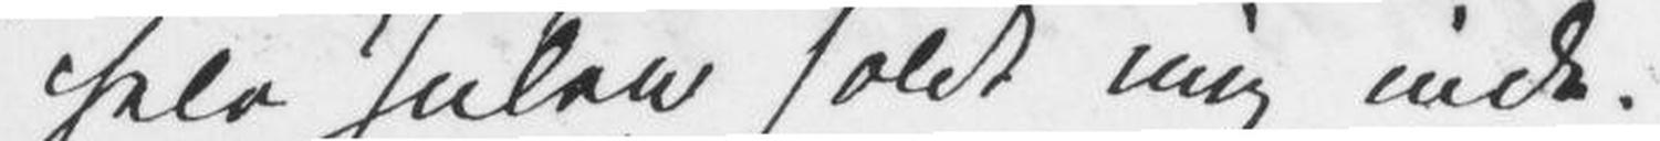hele Julen holdt mig inde.
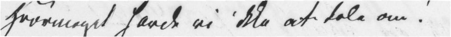Hvormeget havde vi ikke at tale om!
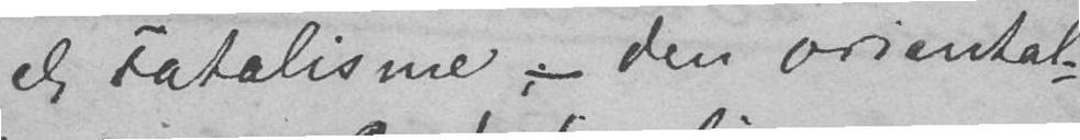el Fatalisme – den oriental-
